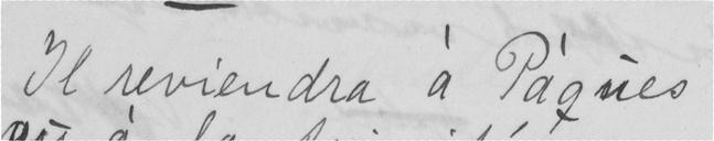Il reviendra á Páques
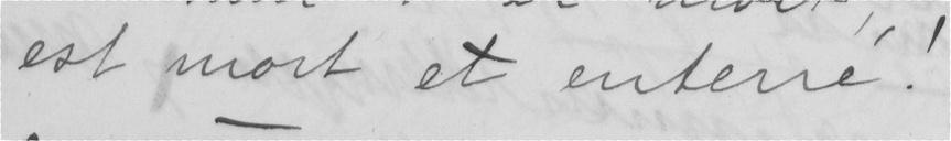est mort et enterré!
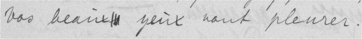vos beairx yeux nont pleurer.
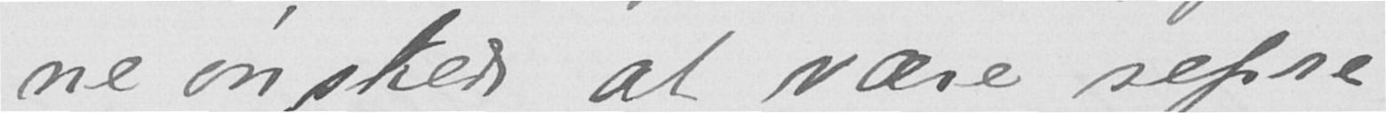ne ønskede at være repre-
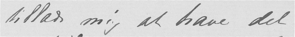tillade mig at have det
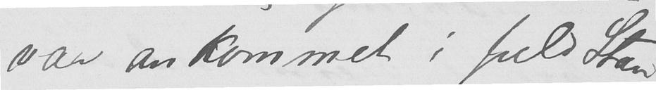var ankommet i fuld Stand
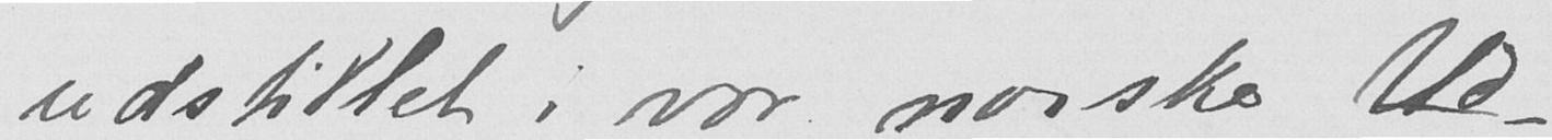udstillet i vor norske Ud-
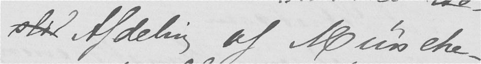stil Afdeling af Münche-
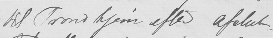til Trondhjem efter afslut-
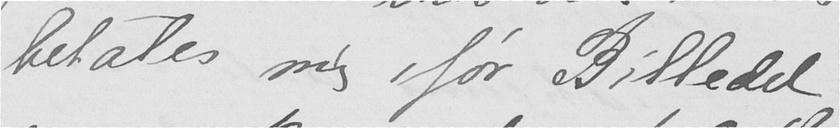betales mig før Billedet
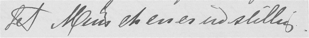tet Münchenerudstilling.
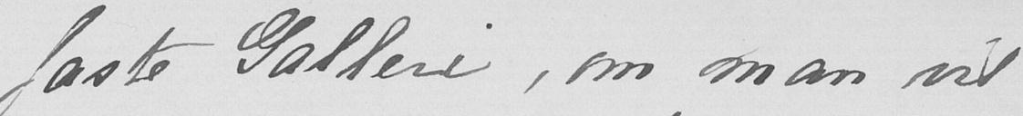faste Galleri, om man vil
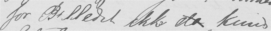for Billedet ikke da kunde
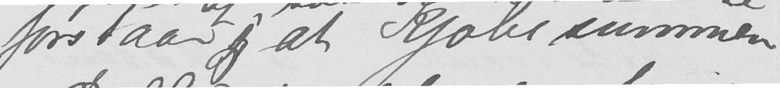forstaar jeg at Kjøbesummen
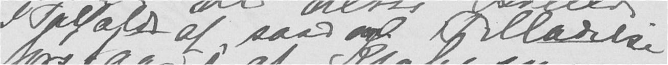I Tilfælde af saadan Tilladelse
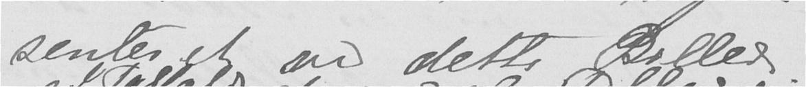senteret ved dette Billede.
